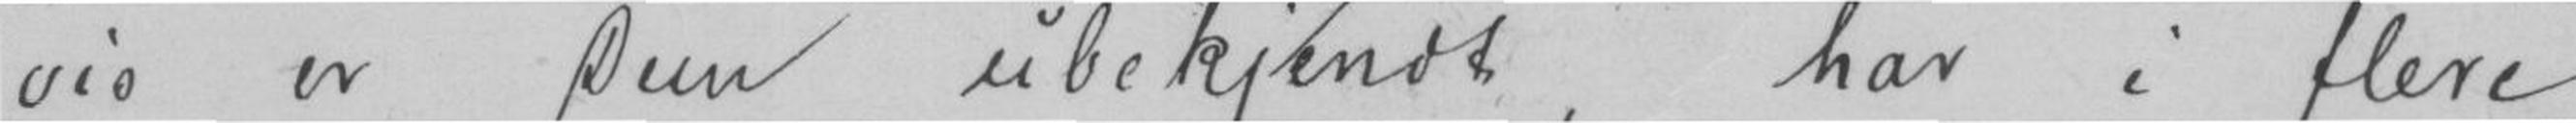vis er Den ubekjendt, har i flere
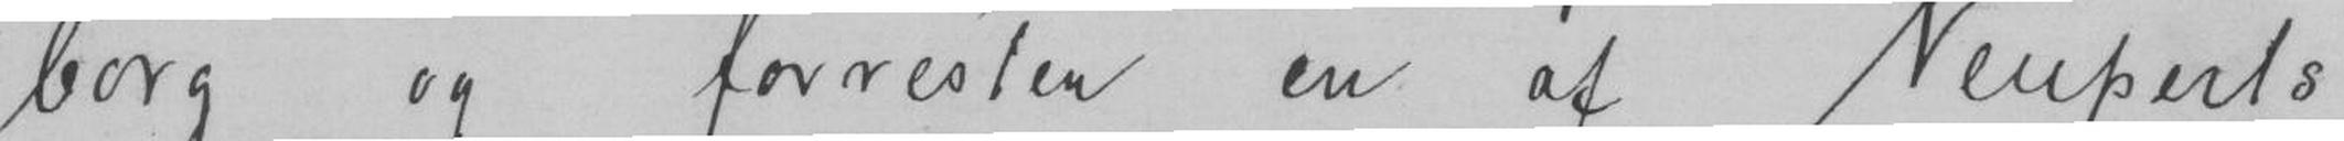borg og forresten en af Neuperts
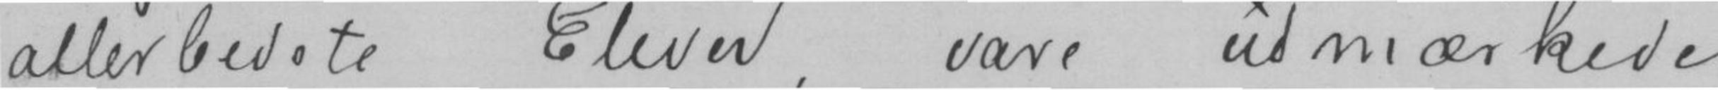allerbedste Elever, være udmærkede
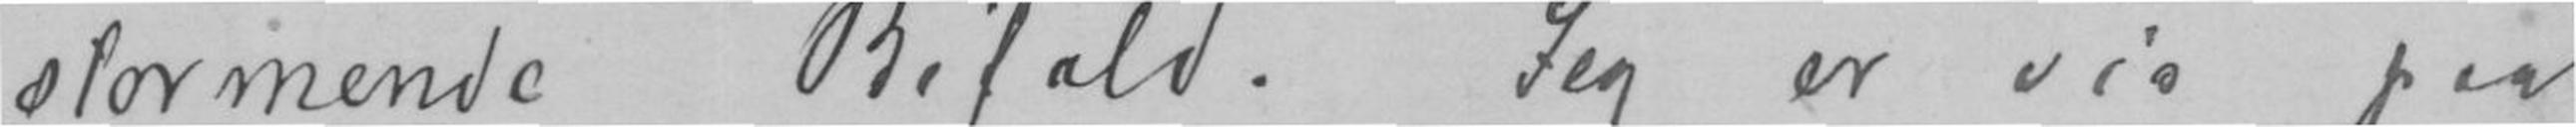stormende Bifald. jeg er vis paa
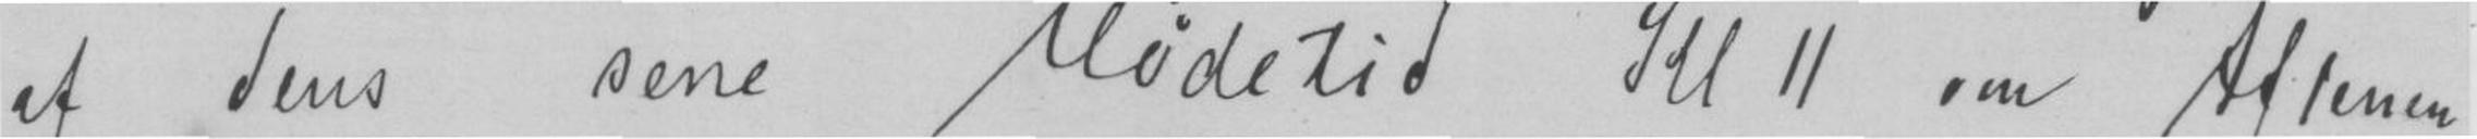af dens sene Mødetid JU 11 om Aftenen
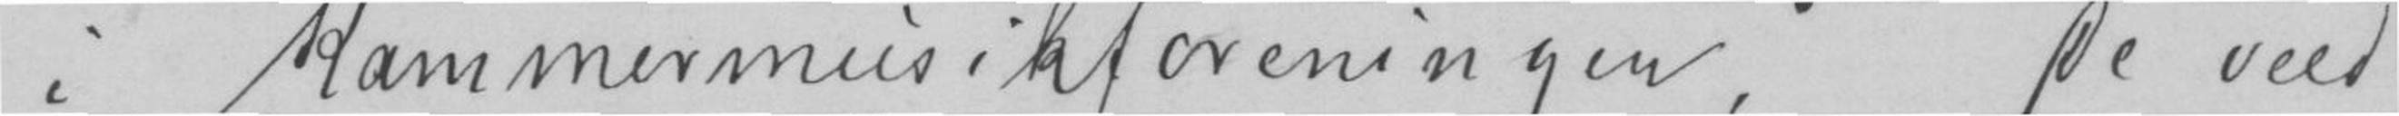i Kammermusikforeningen, De veed
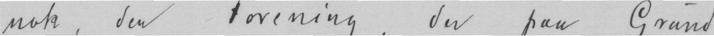nok, den Forening, der paa Grund
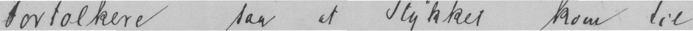Fortolkere, saa et Stykke kom til
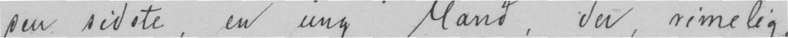den sidste, en ung Mand, der rimelig
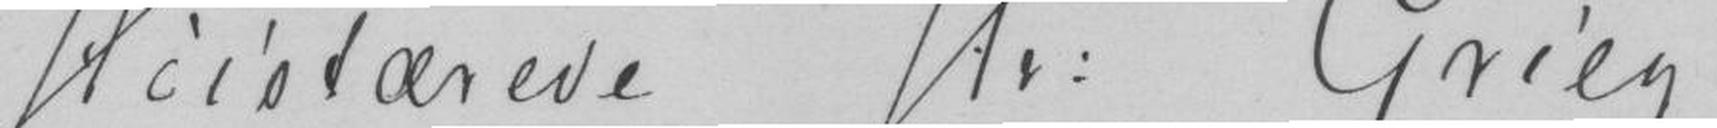Høistærede Hr. Grieg
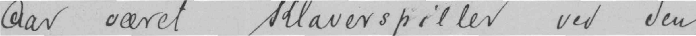Aar været Klaverspiller ved den
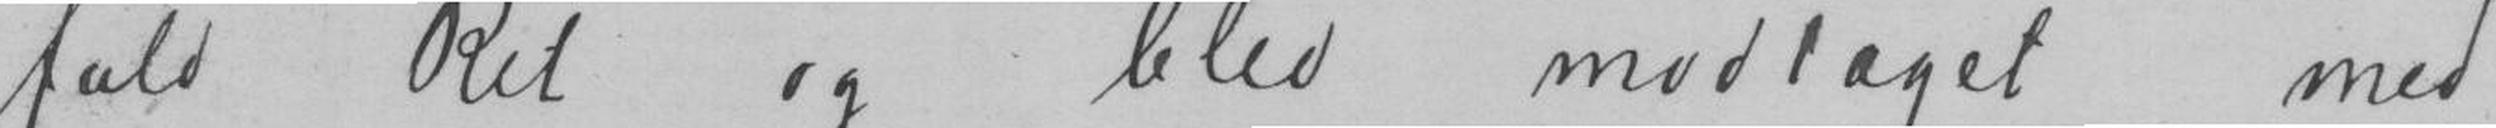fald Ret og blev modtaget med
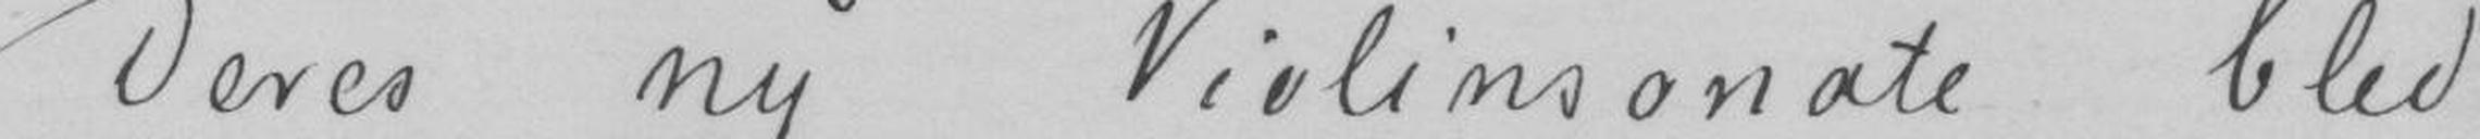Deres nu Violinsonate blev
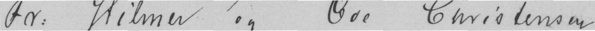Fr. Hilmer og Øde Christensen
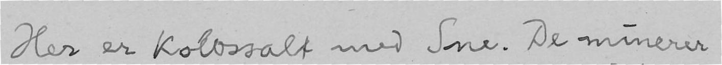Her er kolossalt med Sne. De minerer
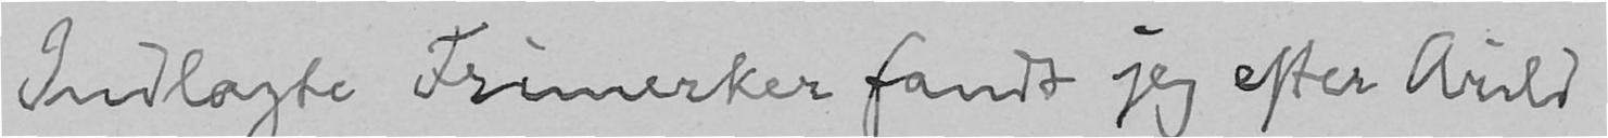Indlagte Frimerker fandt jeg efter Arild
